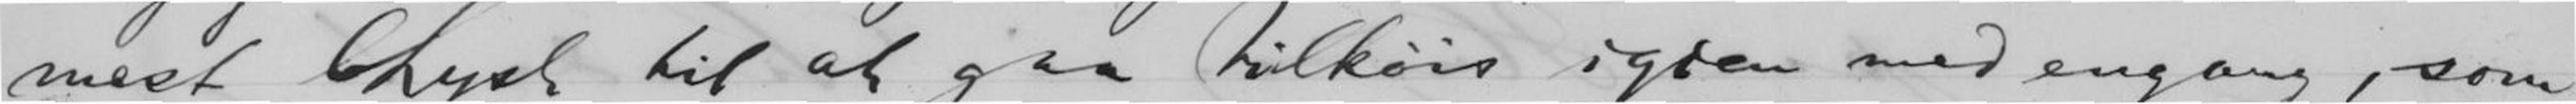mest Lyst til at gaa tilkøis igjen med engang, som
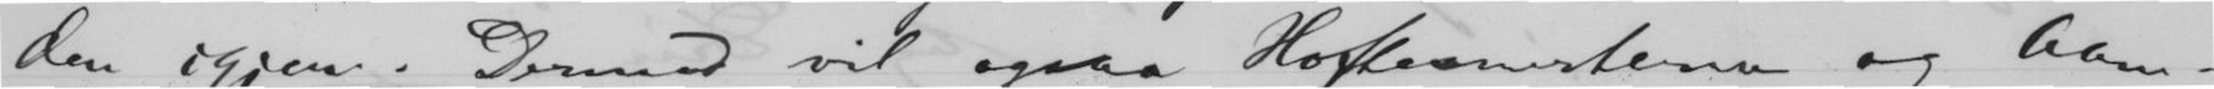den igjen. Dermed vil ogsaa Hoftesmerterne og Aan-
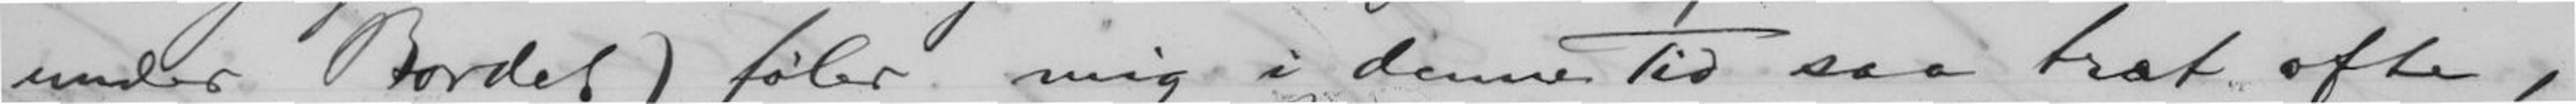under Bordet) føler mig i denne Tid saa træt ofte,
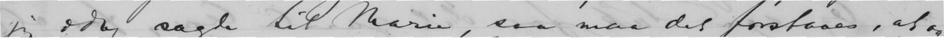jeg idag sagde til Marie, saa maa det forstaaes, at og-
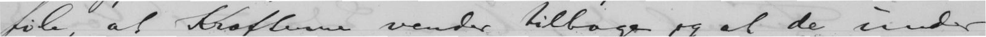føle, at Kræfterne vender tilbage og at de under
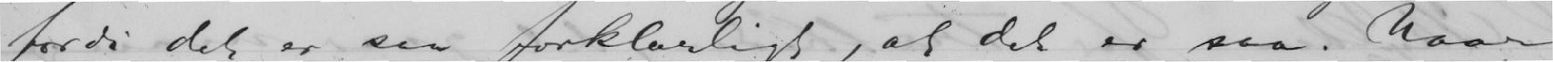fordi det er saa forklarligt, at det er saa. Naar
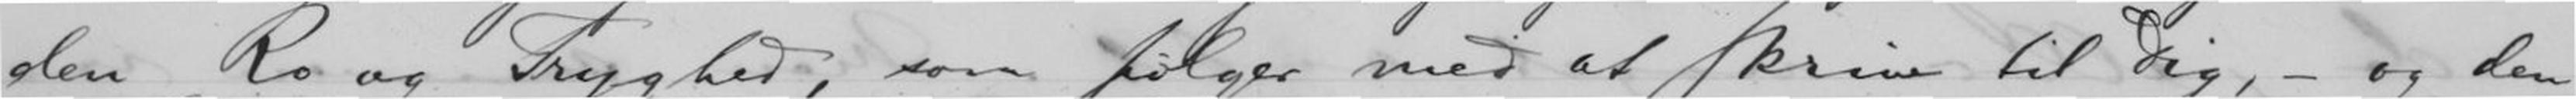den Ro og Tryghed, som følger med at skrive til Dig, - og den
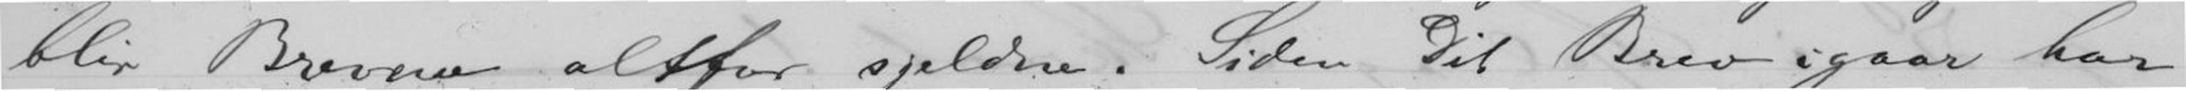blir Brevene altfor sjeldne. Siden Dit Brev igaar har
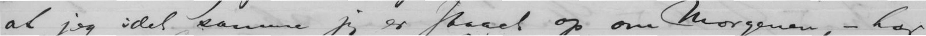at jeg idet samme jeg er staaet op om Morgenen, - har
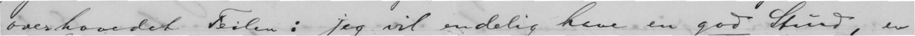overhovedet Feilen: jeg vil endelig have en god Stund, en
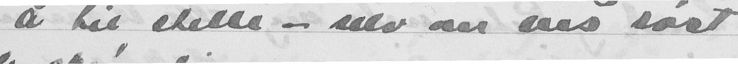å tie stille - selv om ens røst
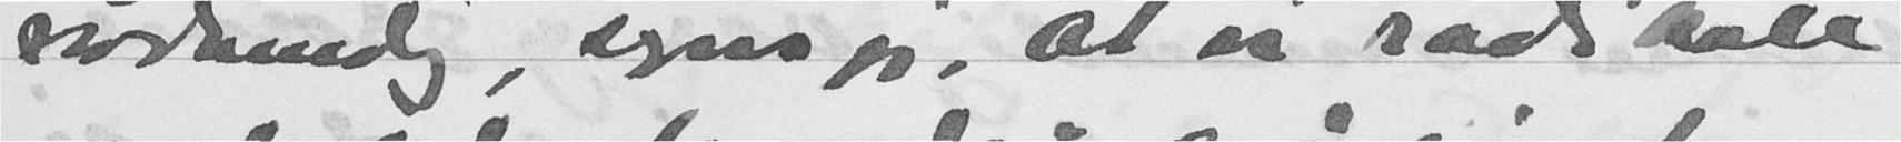nødvendig, syns jeg, at vi radikale
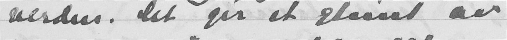verden. Det gir et glimt av
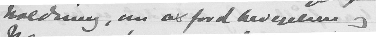holdning, om oxfordbevegelsen og
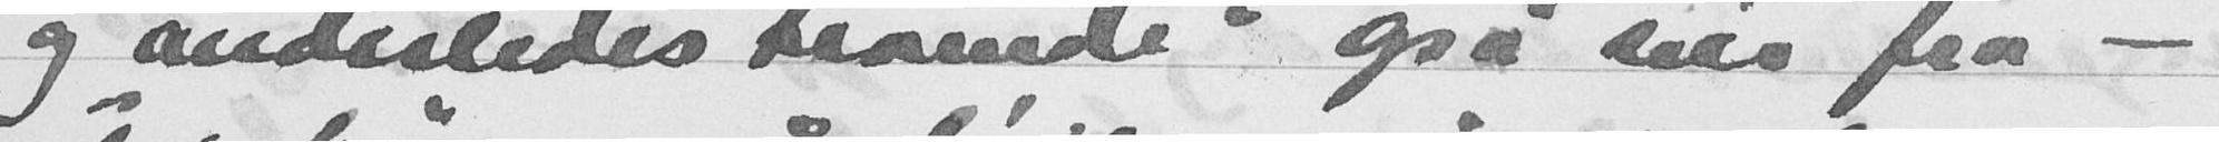og anderledes troende også sier fra -
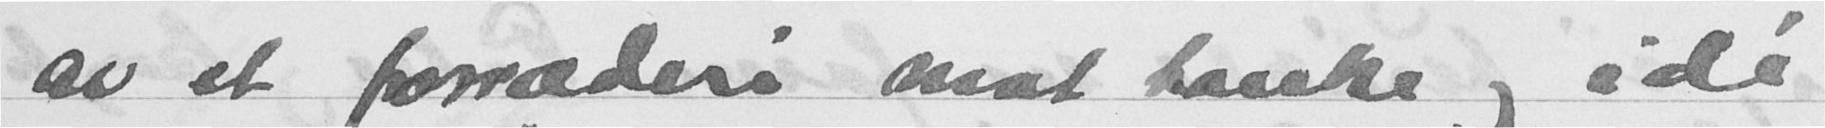av et forræderi mot tanke og idé
